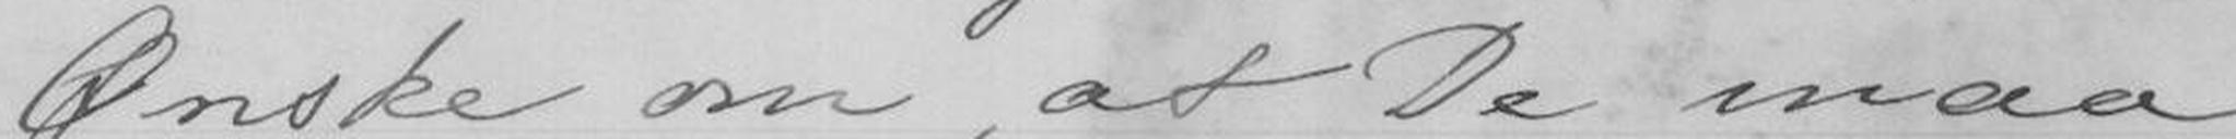Ønske om, at De maa
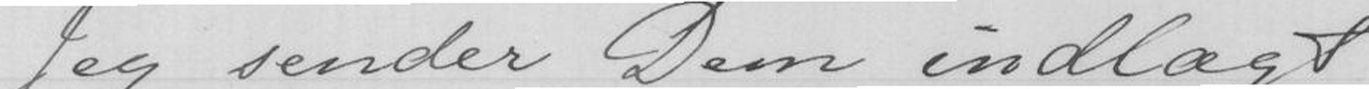Jeg sender Dem indlagt
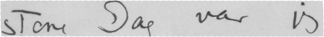store Dag var jeg
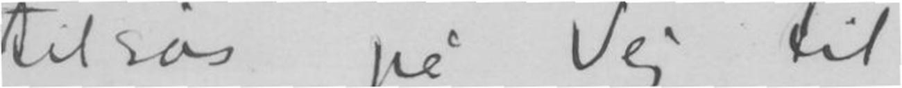tilsøs på Vej til
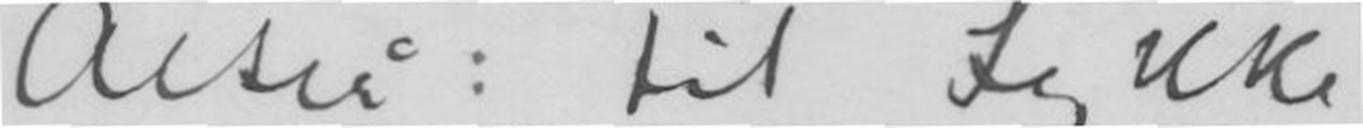Altså: Til Lykke
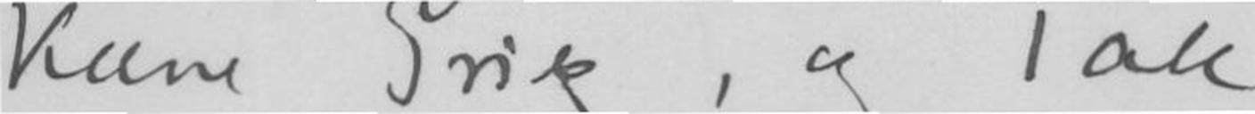Kære Grieg, og Tak
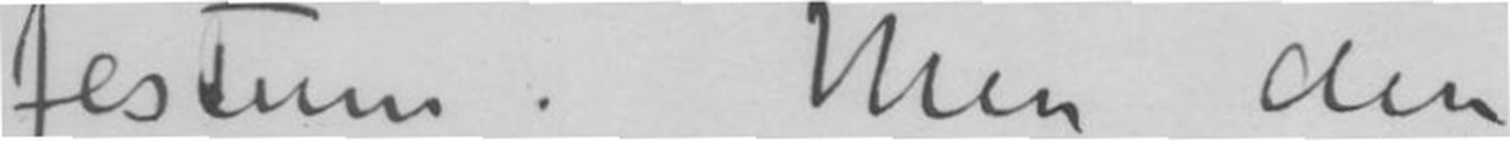festum. Men den
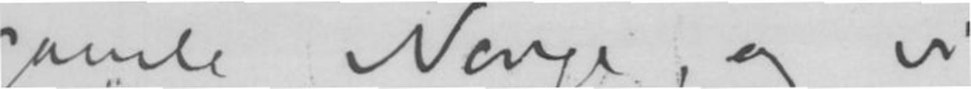gamle Norge, og vi
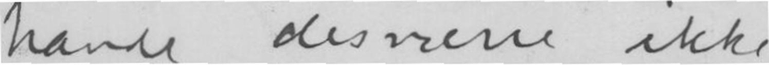havde desværre ikke
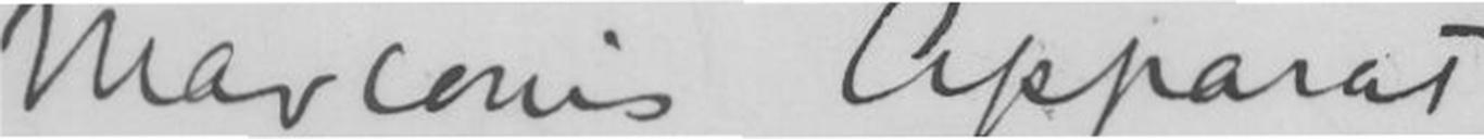Marconis Apparat
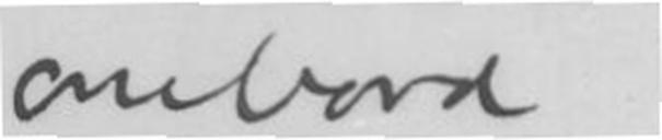ombord.
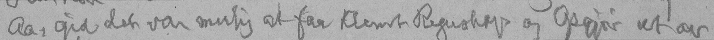Aa, gid det var mulig at faa klemt Regnskap og Opgjør ut av
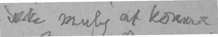ikke mulig at komme
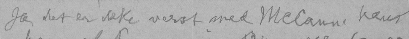Ja det er ikke verst med McCann. Han
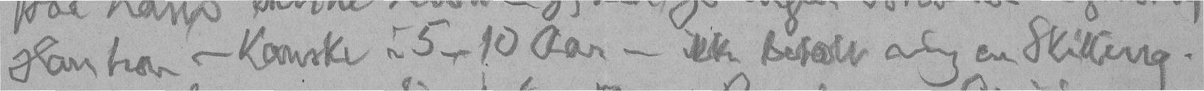Han har - kanske i 5-10 Aar - ikke betalt mig en Skilling.
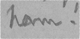ham!
